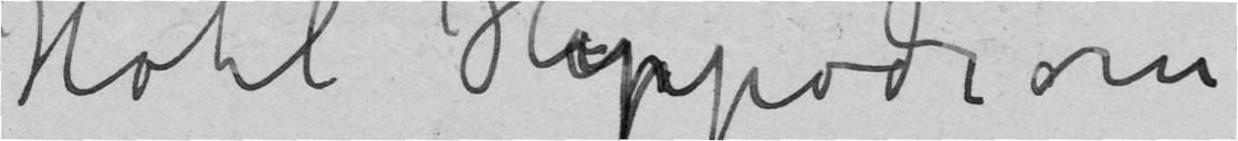Hotel Hippodrom
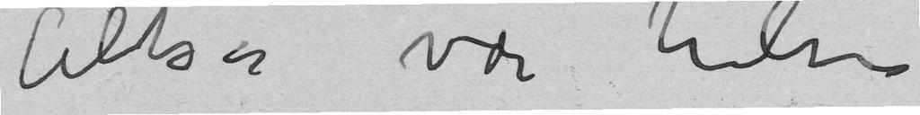Altså vor hilsen
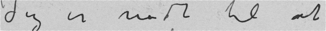Jeg er nødt til at
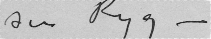sin Ryg –
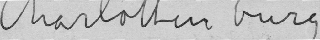Charlottenburg
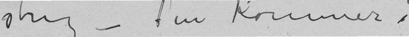streg – den kommer!
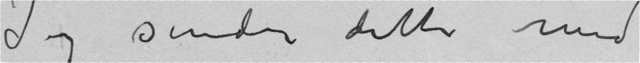Jeg sender dette med
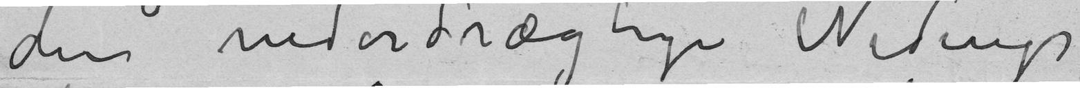den nederdrægtige Nidings
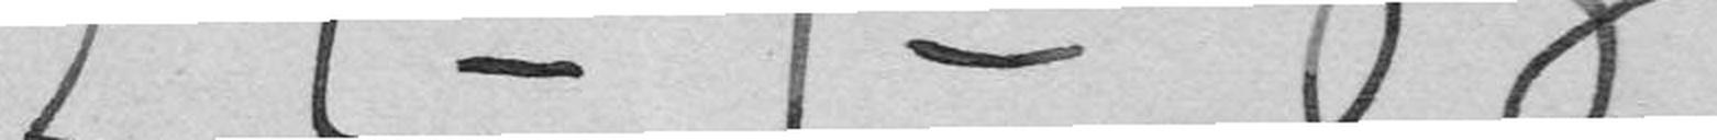22-1-08
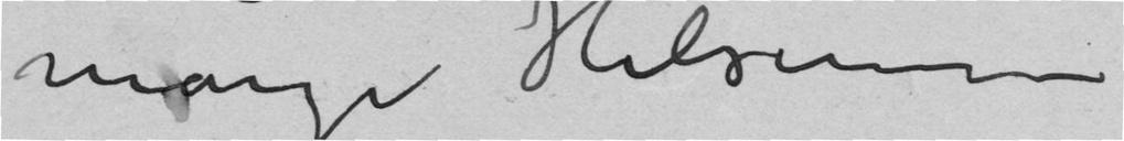mange Hilsener
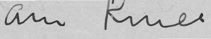Am Knie
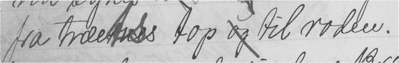fra træets top og til roden.
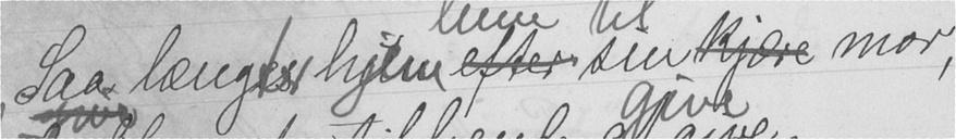Saa længtes hjem hun til sin mor,
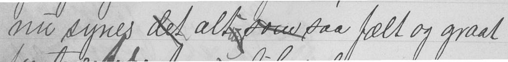nu synes det alt, som saa fælt og graat
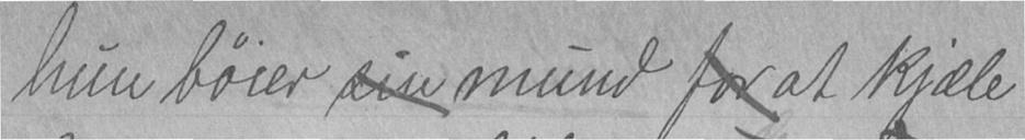hun bøier sin mund for at kjæle
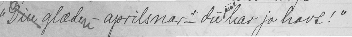"Din glæden, - aprilsnar - du just har jo havt!"
